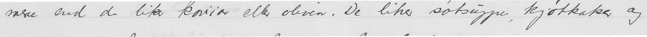mere end de liker kaviar eller oliven. De liker søtsuppe, kjøtkaker og
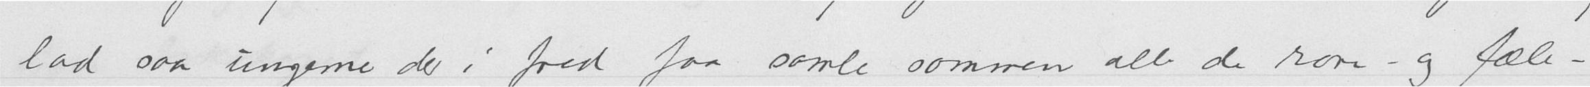lad saa ungerne der i fred faa samle sammen alle de rare – og fæle –
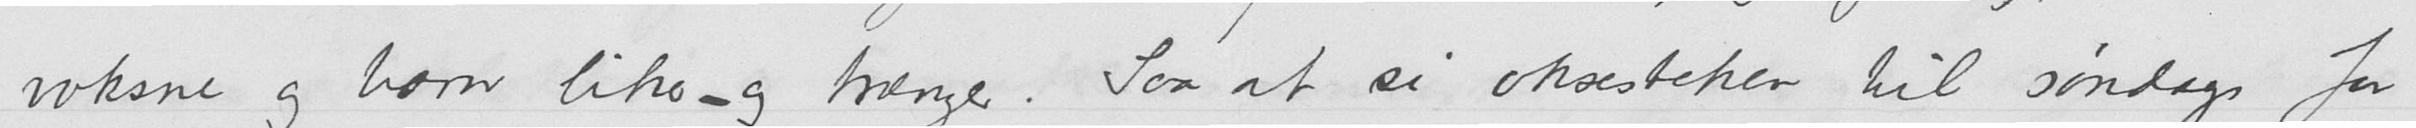voksne og barn liker – og trænger. Saa at si oksesteken til søndags for
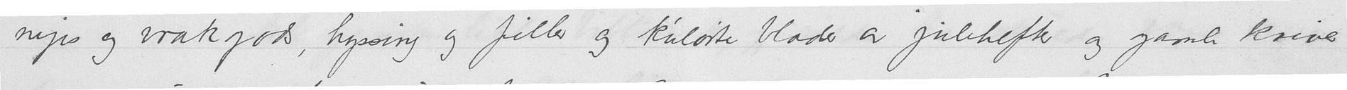nips og vrakgods, hyssing og filler og kulørte blader av julehefter og gamle kniver
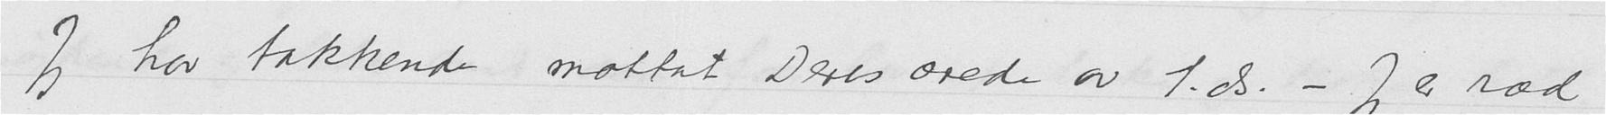Jeg har takkende mottat Deres ærede av 1.ds. - Jeg er ræd
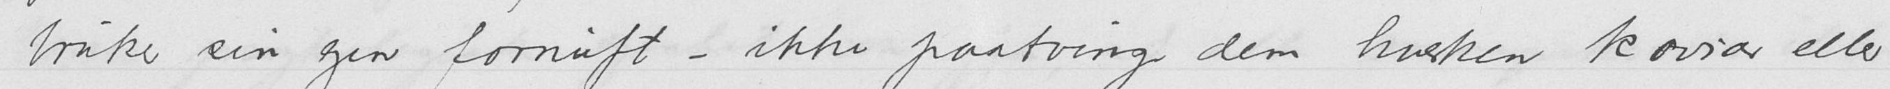bruker sin egen fornuft – ikke paatvinge dem hverken kaviar eller
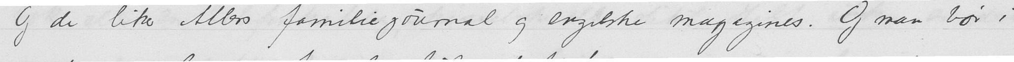Og de liker Allers familiejournal og engelske magazines. Og man bør i
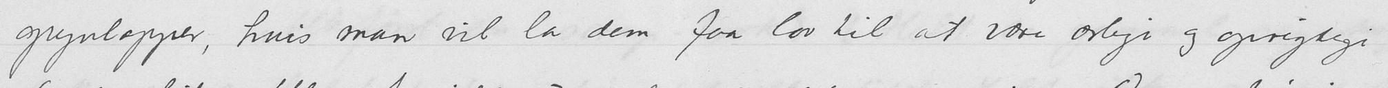grynlapper, hvis man vil la dem faa lov til at være ærlige og oprigtige
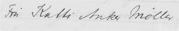Fru Katti Anker Møller
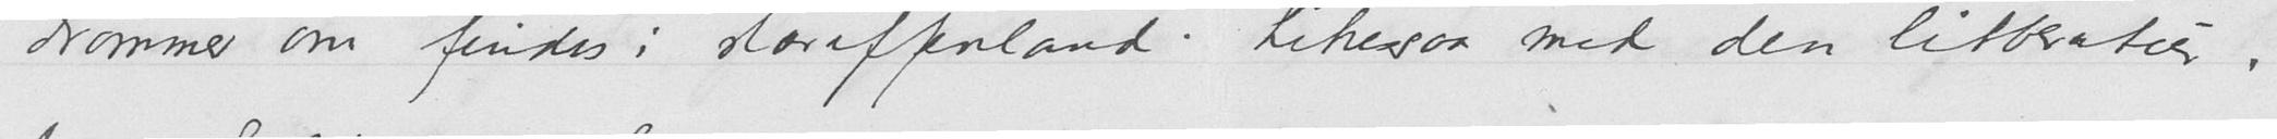drømmer om findes i slaraffenland. Likesaa med den litteratur
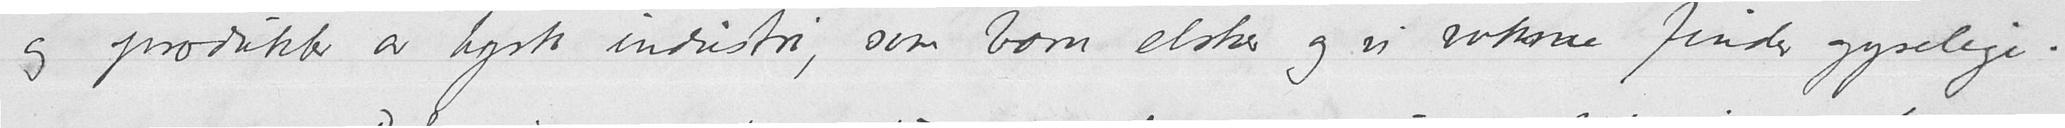og produkter av tysk industri, som barn elsker og vi voksne finder gyselige.
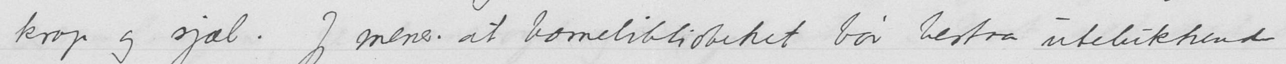krop og sjæl. Jeg mener at barnebiblioteket bør bestaa utelukkende
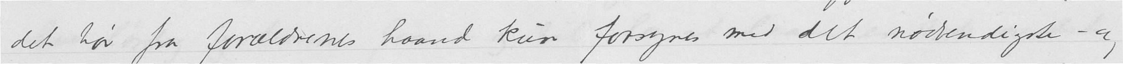det bør fra forældrenes haand kun forsynes med det nødvendigste – og
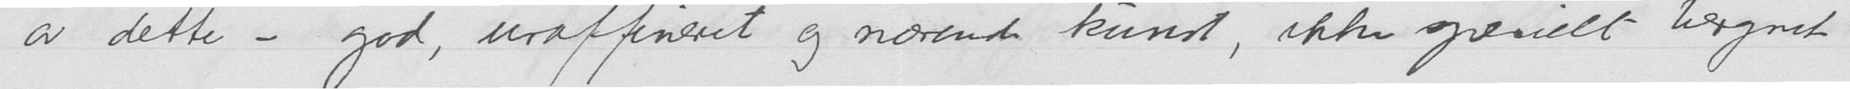av dette – god, uraffineret og nærende kunst, ikke spesielt beregnet
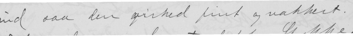ind saa den virked fint og vakkert.
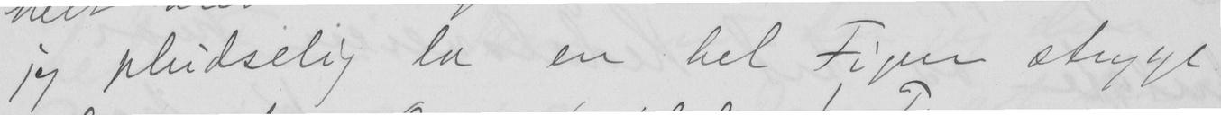jeg pludselig la en hel Figur stryge
